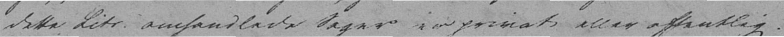dette Litr. omhandlede Sager er privat eller offentlig.
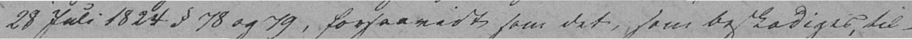28 Juli 1824 § 78 og 79, forsaavidt som det, som beskadiges, til-
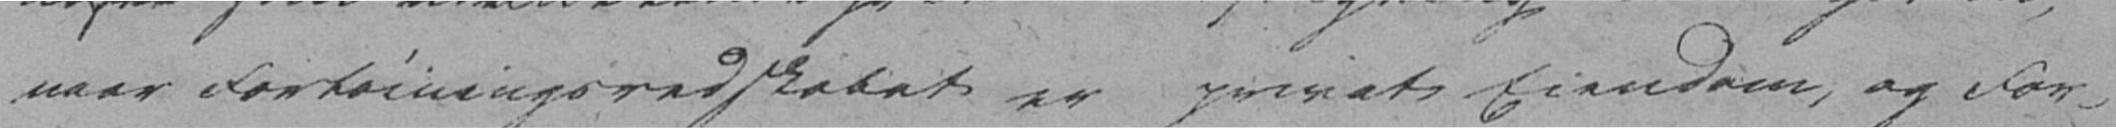naar Forføiningsredskabet er privat Eiendom, og For-
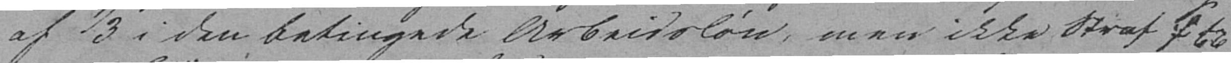af 1/3 i den betingede Arbeidsløn, men ikke Straf f. Ex.
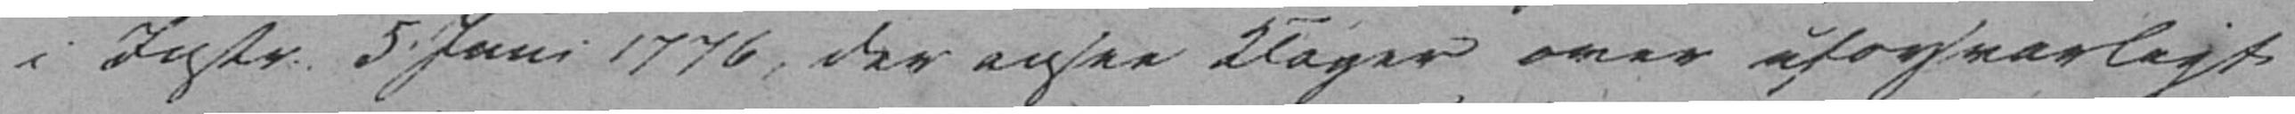i Instr. 5. Juni 1776, der ansee Klager over uforsvarligt
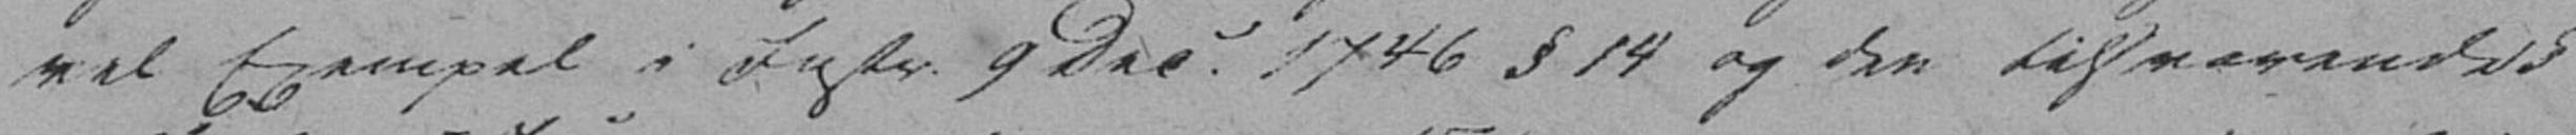vel Exempel i Instr. 9 Dec. 1746 § 14 og den tilsvarende §
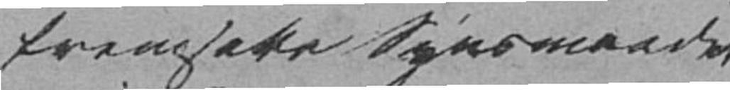fremsatte Synsmaade
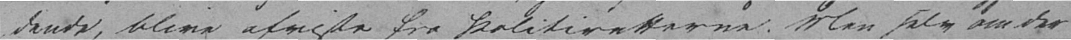dende, blive afviste fra Politiretterne. Men selv om der
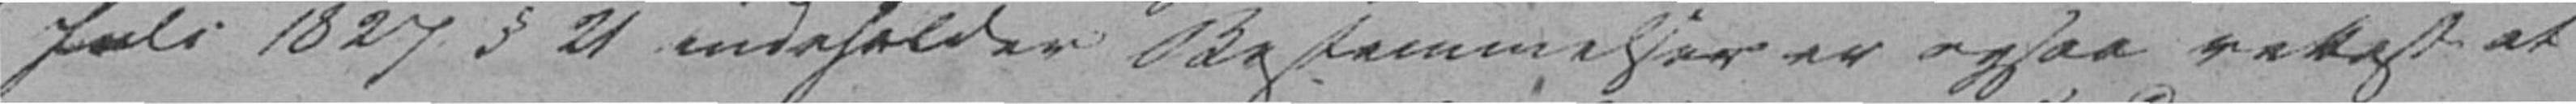Juli 1827 § 21 indeholder Bestemmelser er ogsaa rettest at
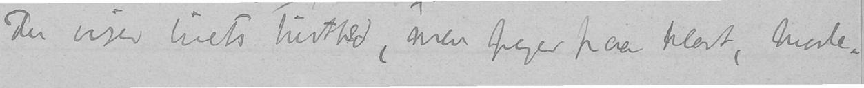Du viser livets tristhed, men peger paa klart, hvorle-
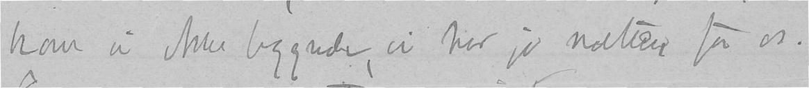kan vi ikke begynde, vi har jo natten for os.
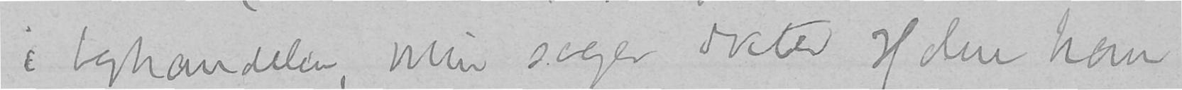i boghandelen, min svoger doctor Holm kom
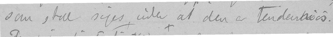som skal siges uden at den er tendentiøs.
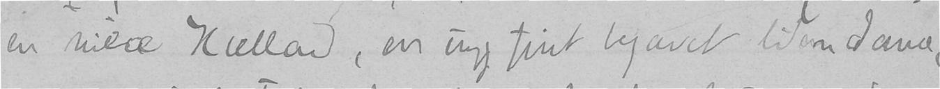en niece Kielland, en ung fint begavet liden dame,
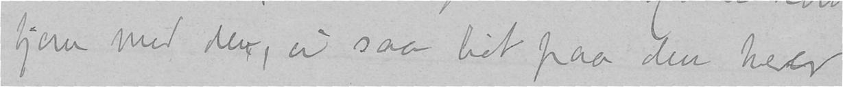hjem med den, vi saa lidt paa den hver
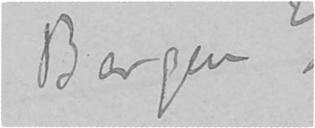Bergen,
